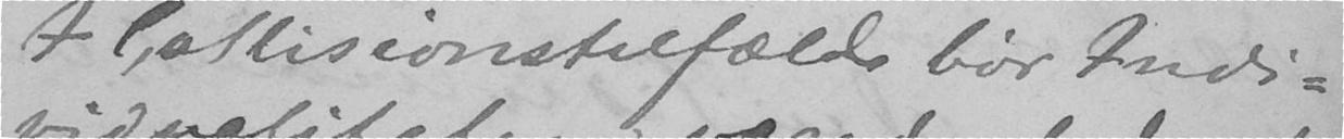I Collisionstilfælde bør Indi-
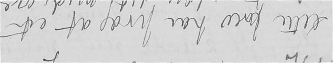Dette brev har præg af et
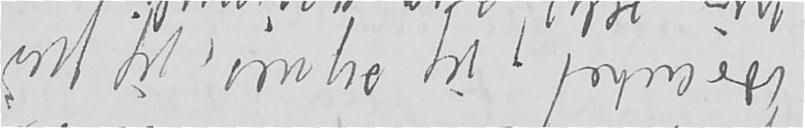bærenhed, jeg synes, jeg gjør
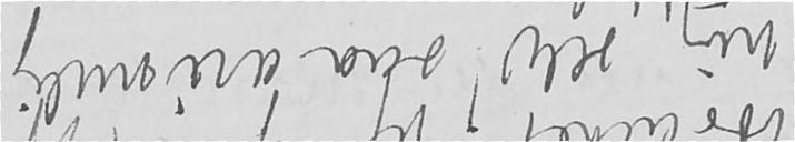mig selv saa urimelig
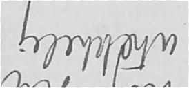utækkelig
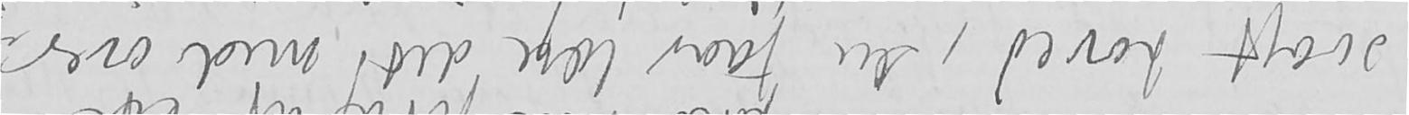svagt hoved, Du faar læse det med over-
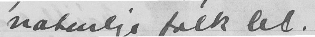naturlige folk lel.
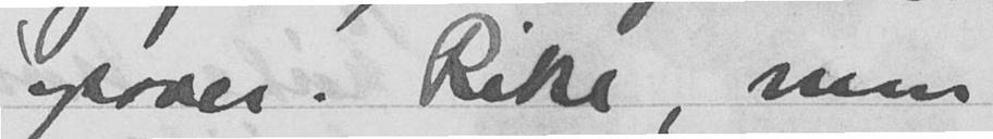opover. Rike, men
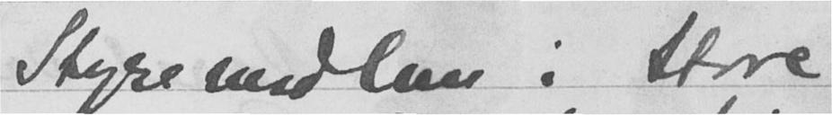Styremedlem i Store
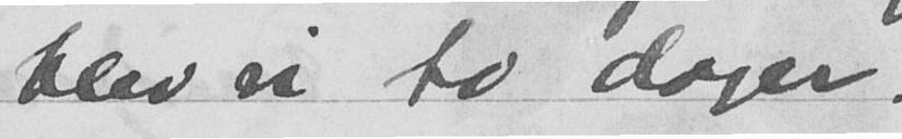blev vi to dager.
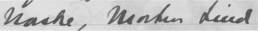Norske, Morten Lind
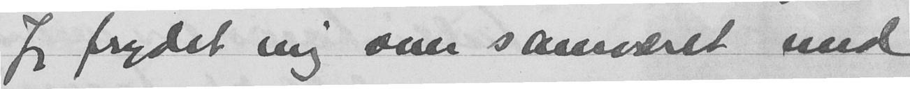Jeg frydet mig over samværet med
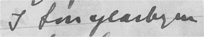I Longyearbyen
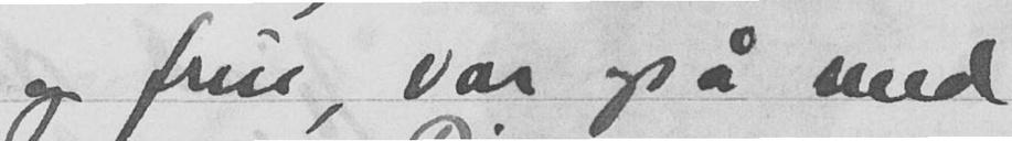og frue, var også med
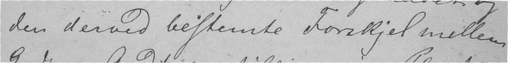den derved bestemte Forskjel mellem
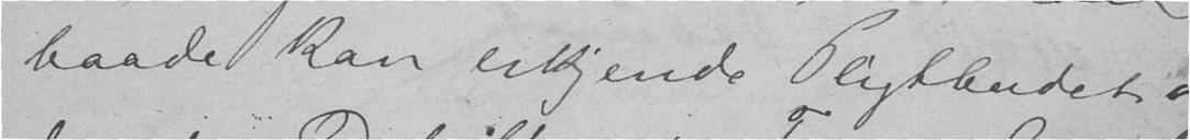baade kan erkjende Pligtbudet og
